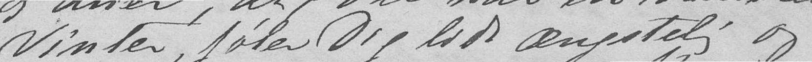Vinter, føler Dig lidt ængstelig og
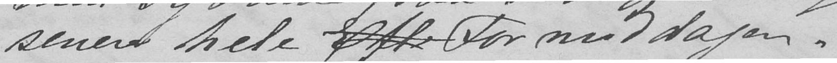senere hele Efte Formiddagen.
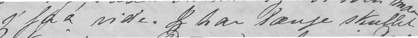jeg faa vide. Jeg har længe skullet
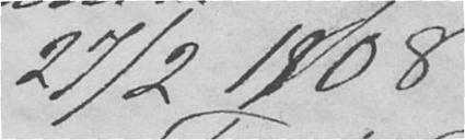27/2 1908
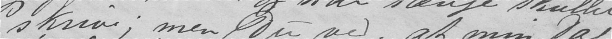skrive; men Du ved at min Dag
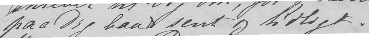paa Dig baade sent og tidligt.
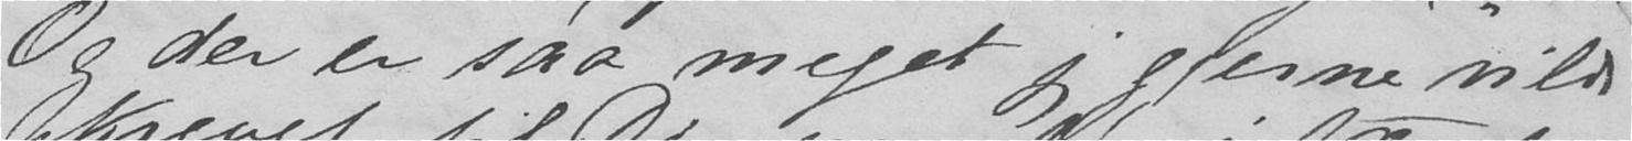Og der er saa meget jeg gjerne vilde
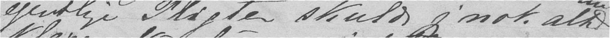egentlige Pligter skulde jeg nok altid
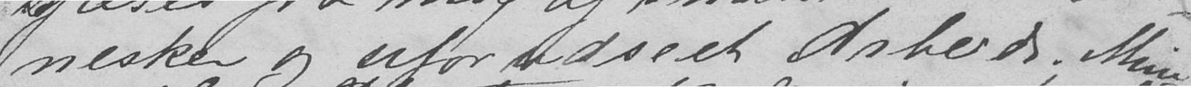nesker og uforudseet Arbeide. Mine
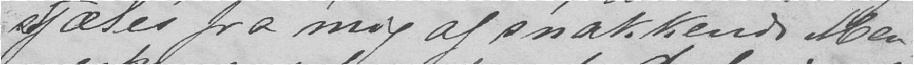stjæles fra mig af snakkende Men-
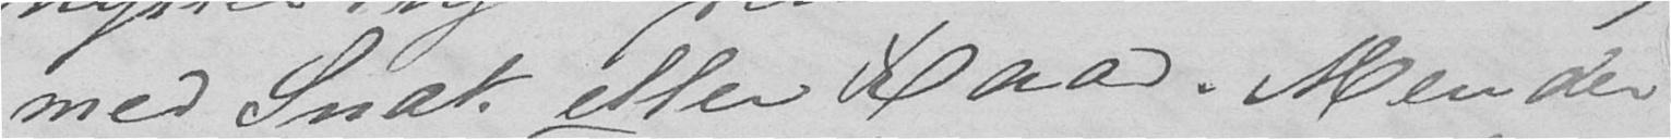med Snak eller Raad. Men der
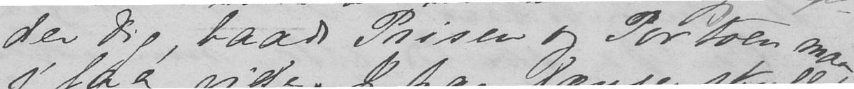der dig, baade Prisen og Portoen maa
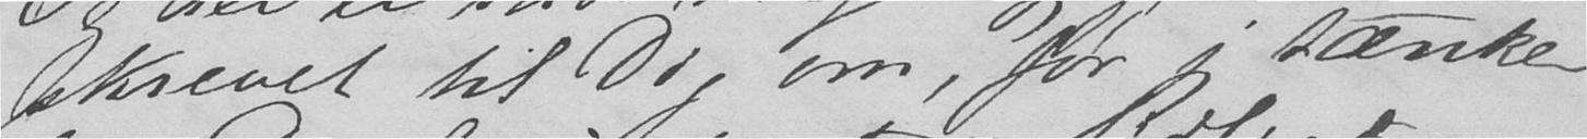skrevet til Dig om, for jeg tænker
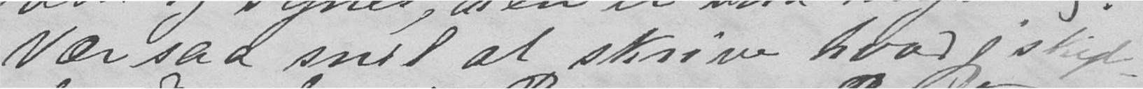Vær saa snil at skrive hvad jeg skyl-
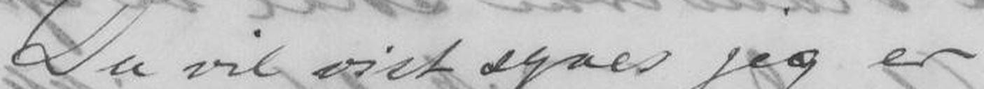Du vil vist synes jeg er
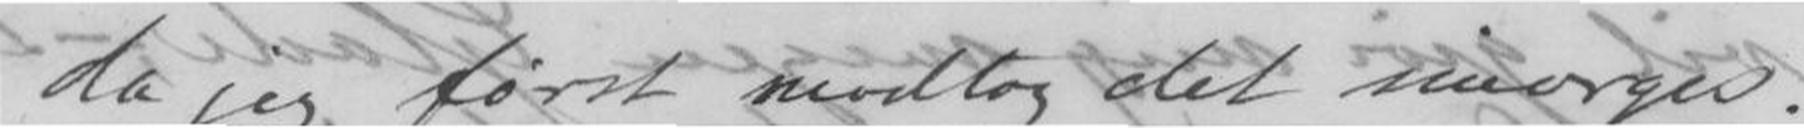da jeg først modtog det imorges.
